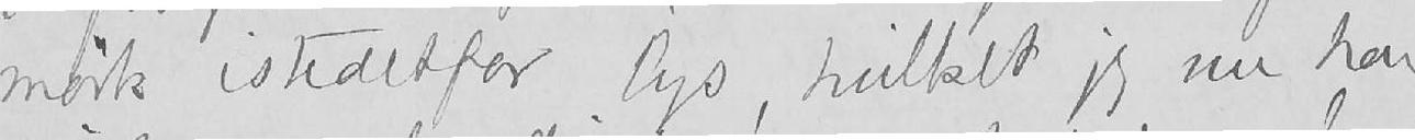mørk istedetfor lys, hvilket jeg nu har
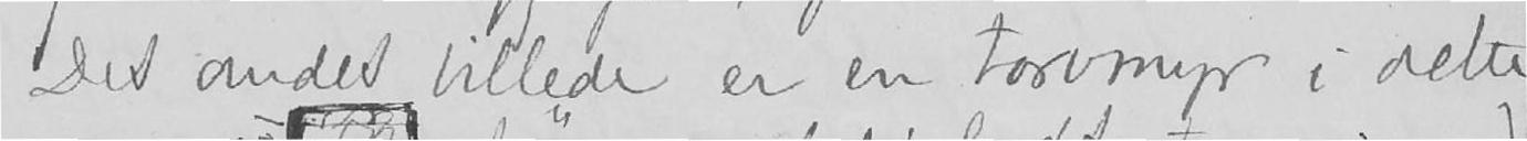Det andet billede er en torvmyr i dette
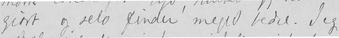giort og selv finder meget bedre. Jeg
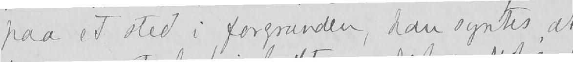paa et sted i forgrunden, han syntes, at
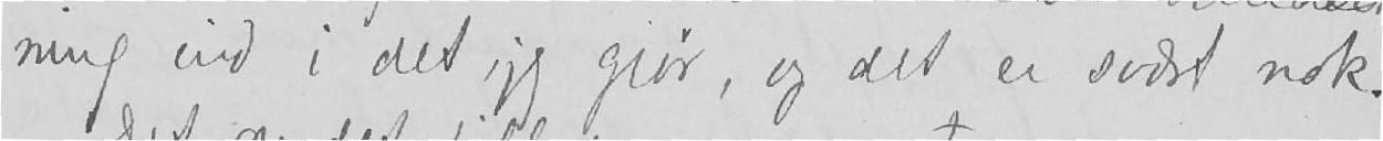ning ind i det jeg giør, og det er svært nok.
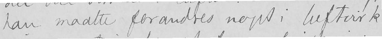han maatte forandres noget i luftvirk-
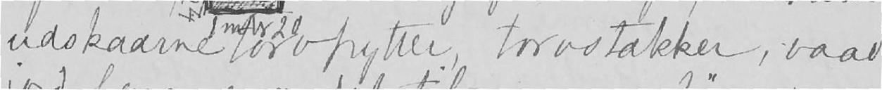udskaarne torvpytter, torvstakker, vaad
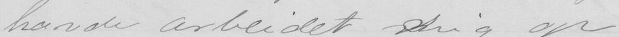havde arbeidet mig op
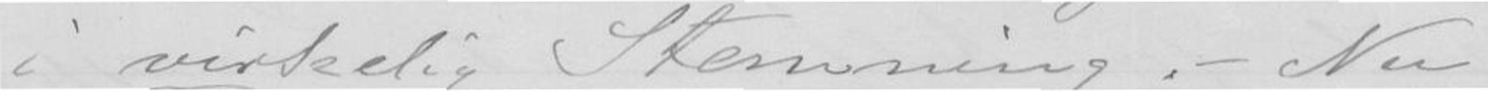i virkelig Stemning. - Nu
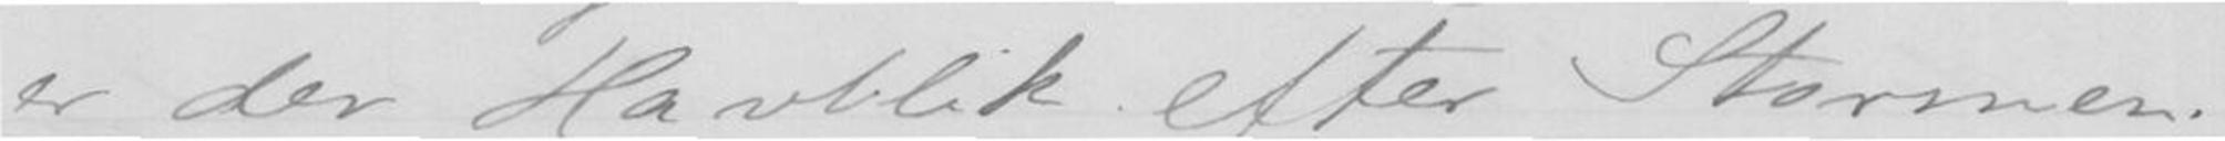er der Havblik efter Stormen.
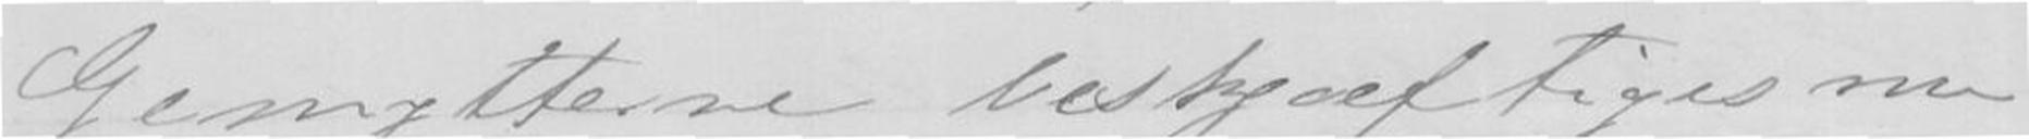Gemytterne beskjæftiges nu
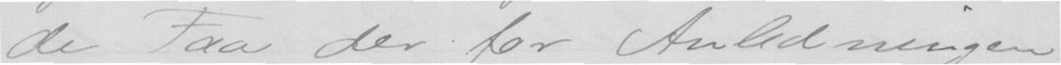de Faa der for Anledningen
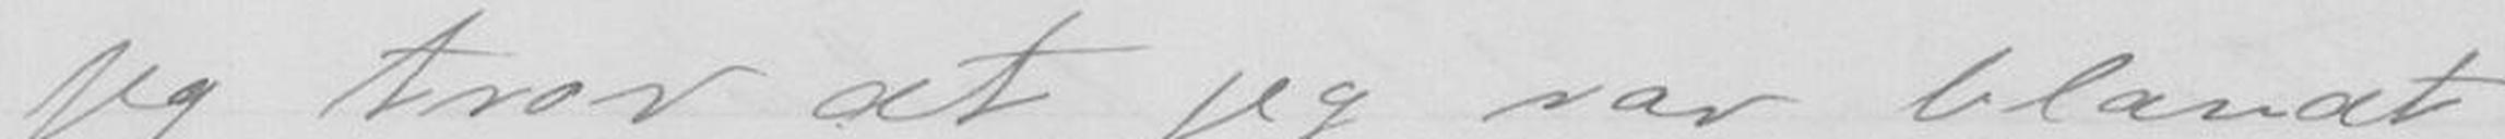jeg tror at jeg var blandt
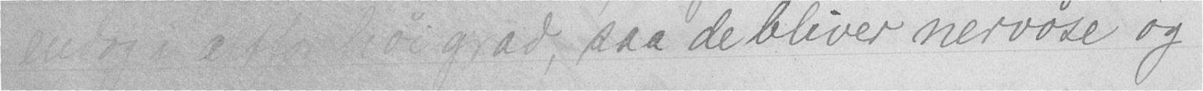endog i altfor høi grad, saa de bliver nervøse og
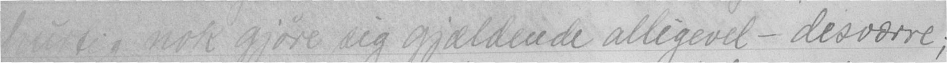hurtig nok gjøre sig gjældende alligevel - desværre;
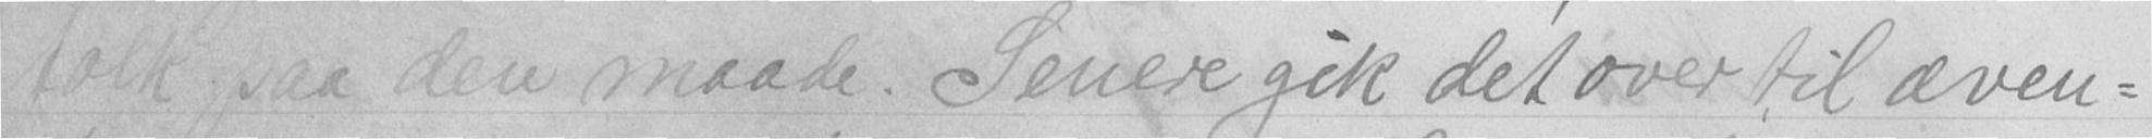folk paa den maade. Senere gik det over til æven-
